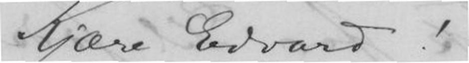Kjære Edvard!
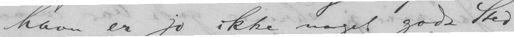havn er jo ikke noget godt Sted
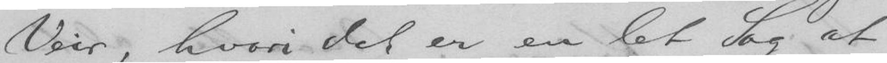Veir, hvori det er en let Sag at
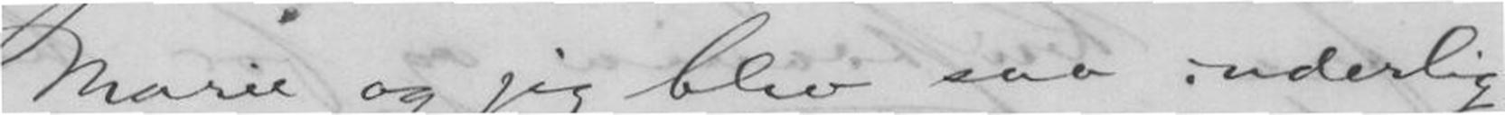Marie og jeg blev saa inderlig
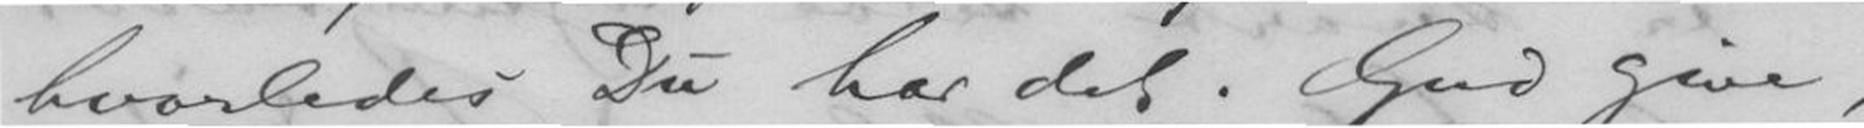hvorledes Du har det. Gud give,
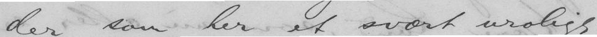der som her et svært uroligt
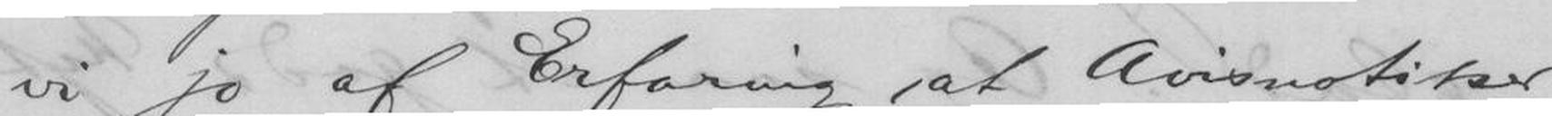vi jo af Erfaring, at Avisnotitser
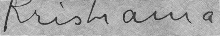Kristiania
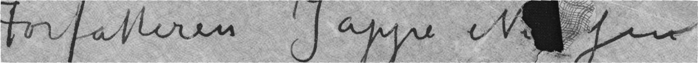Forfatteren Jappe Nisen
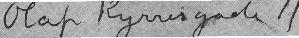Olaf Kyrresgade 11
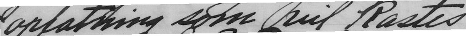opfatning som vil kastes
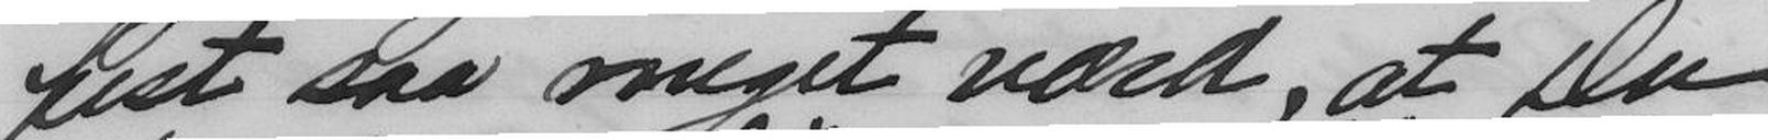fest saa meget værd, at Du
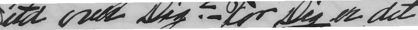ud over Dig? - For Dig er det
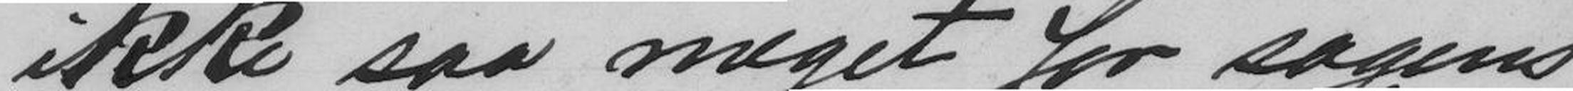ikke saa meget for sagens
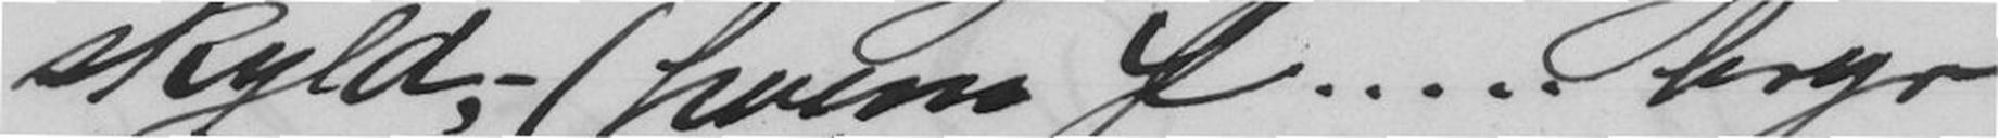skyld, - (hvem f..... bryr
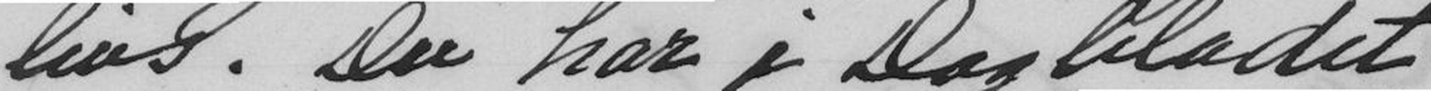livs. Du har i Dagbladet
